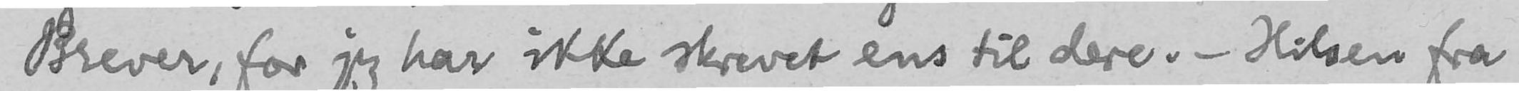Brever, for jeg har ikke skrevet ens til dere. - Hilsen fra
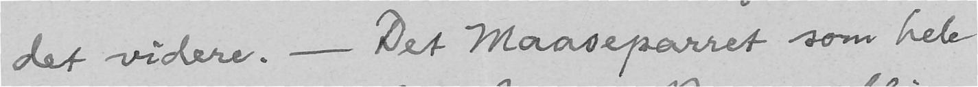det videre. - Det Maaseparret som hele
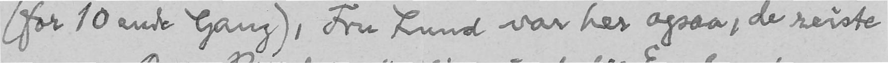(for 10ende Gang), Fru Lund var her ogsaa, de reiste
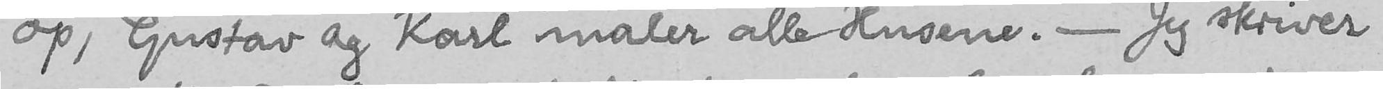op, Gustav og Karl maler alle Husene. - Jeg skriver
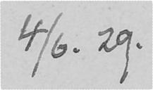4/6. 29.
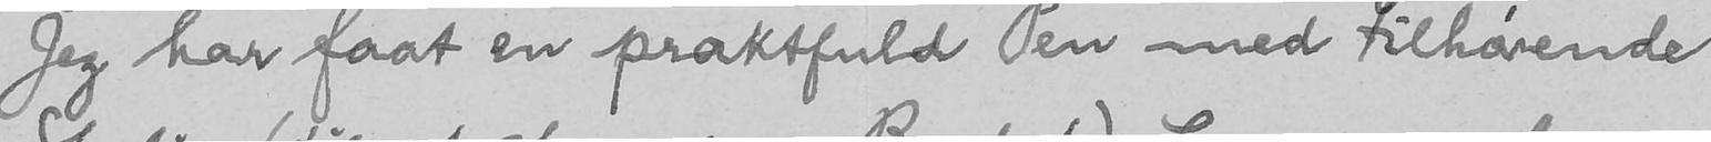Jeg har faat en praktfuld Pen med tilhørende
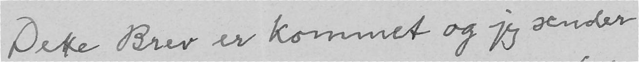Dette Brev er kommet og jeg sender
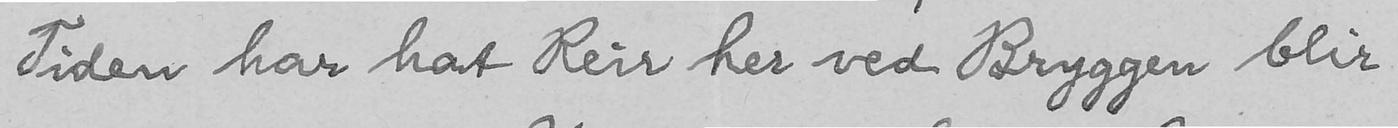Tiden har hat Reir her ved Bryggen blir
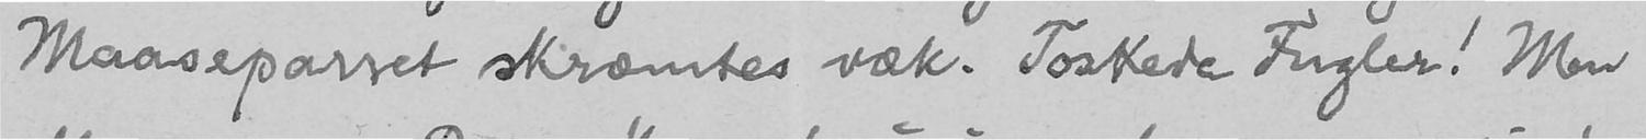Maaseparret skræmtes væk. Toskede Fugler! Men
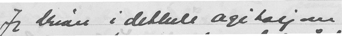Jeg denen i dethele agitasjoner
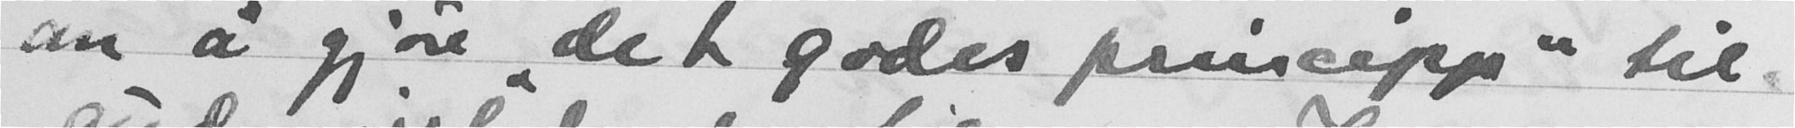om å gjøre "det godes prinsipp" til
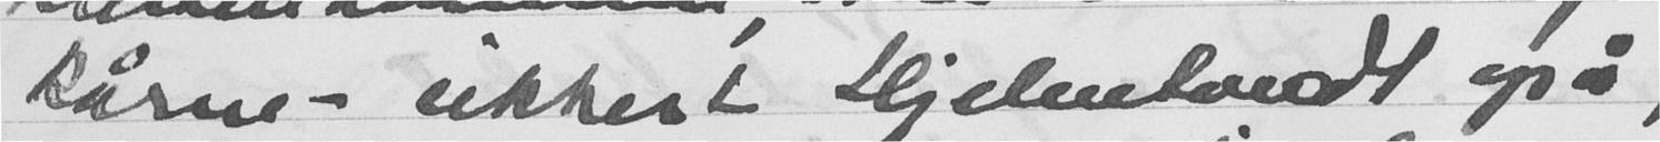kårne - sikkert Hjelmtveidt også,
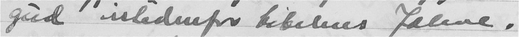gud istedenfor bibelens alene.
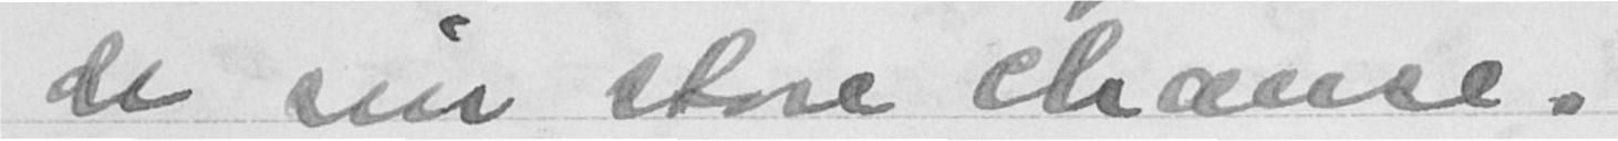de sin store chanse.
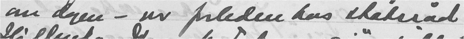om dagen - var forleden hos statsråd
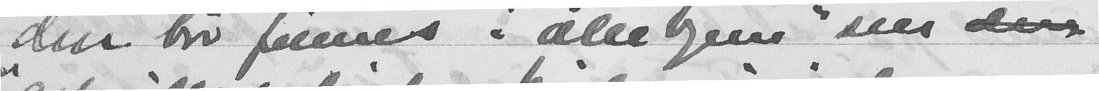"den bør finnes i alle hjem "sier
